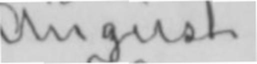August
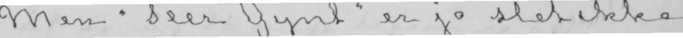Men «Peer Gynt» er jo slet ikke
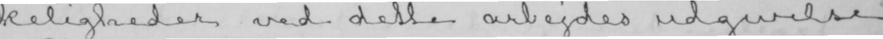keligheder ved dette arbejdes udgivelse
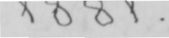1881
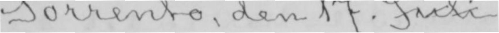Sorrento, den 17. Juli 1881.
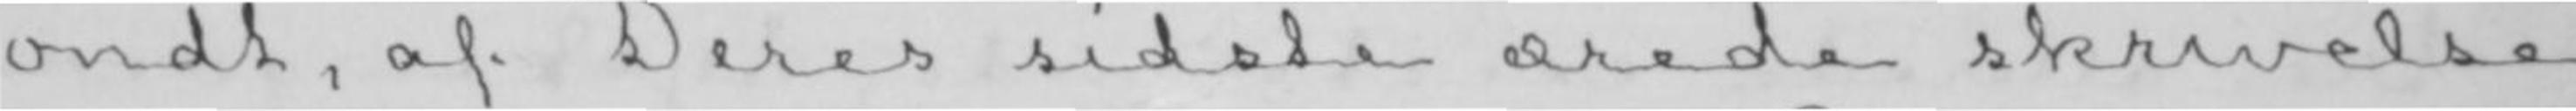ondt, af Deres sidste ærede skrivelse
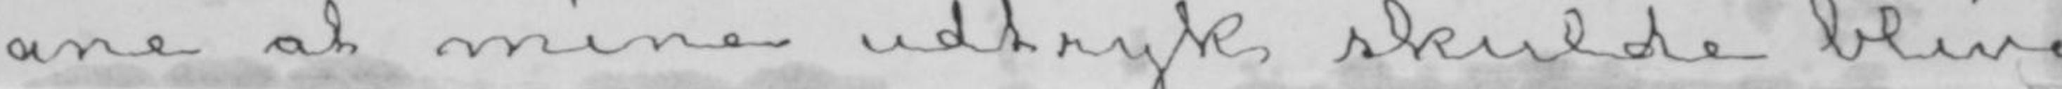ane at mine udtryk skulde blive
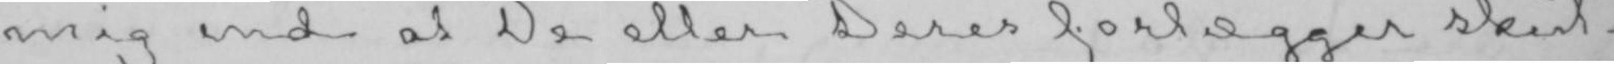mig ind at De eller Deres forlægger skul-
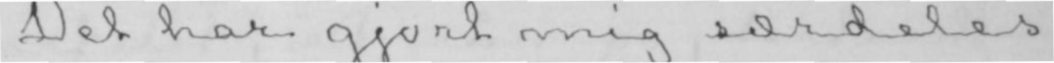Det har gjort mig særdeles
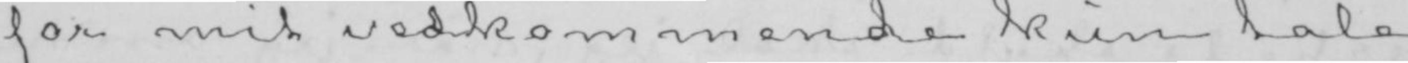for mit vedkommende kun tale
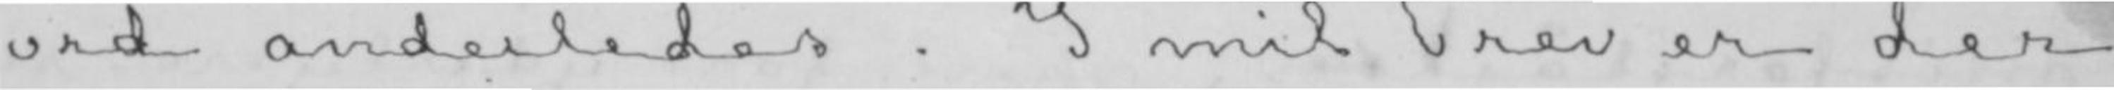ord anderledes. I mit brev er der
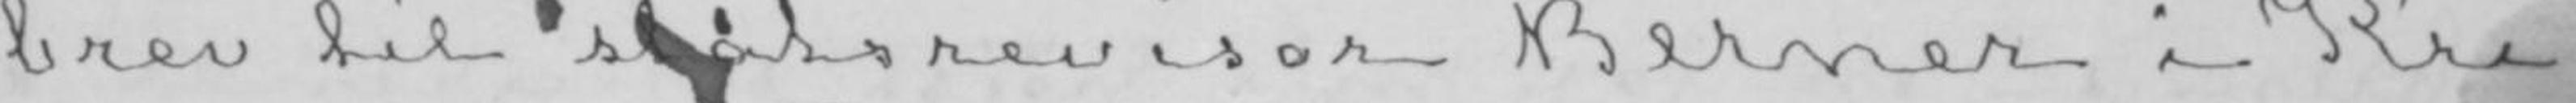brev til statsrevisor Berner i Kri-
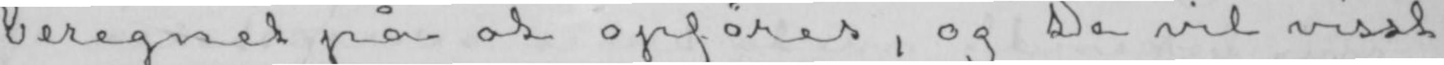beregnet på at opføres, og De vil visst
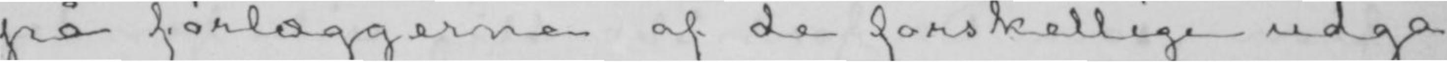på forlæggerne af de forskellige udga-
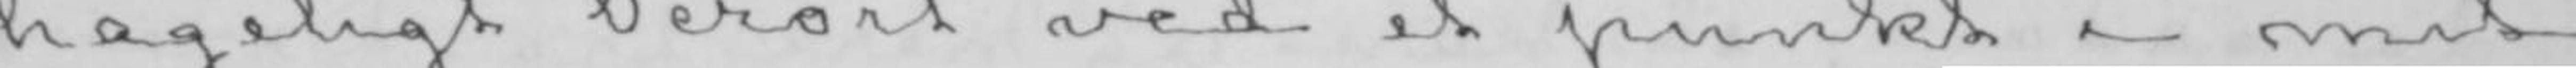hageligt berørt ved et punkt i mit
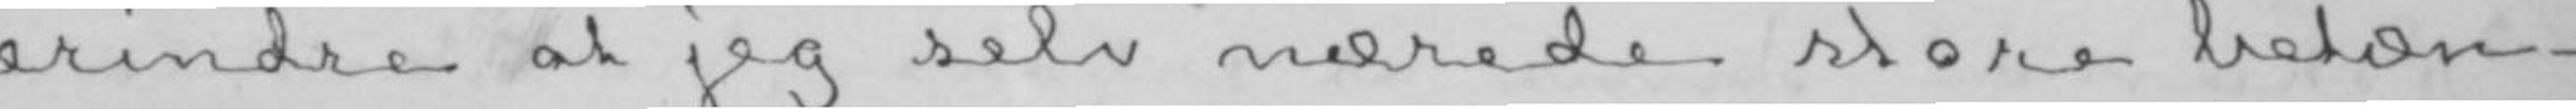erindre at jeg selv nærede store betæn-
